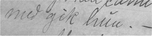med gik hun. -
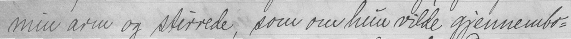min arm og stirrede, som om hun vilde gjennembo-
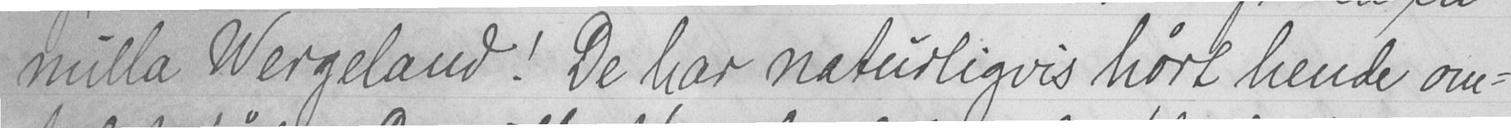milla Wergeland! De har naturligvis hørt hende om-
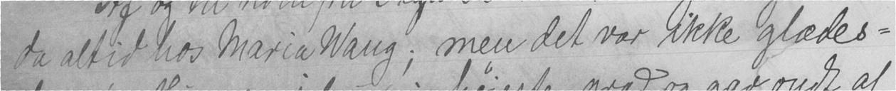da altid hos Maria Wang; men det var ikke glædes-
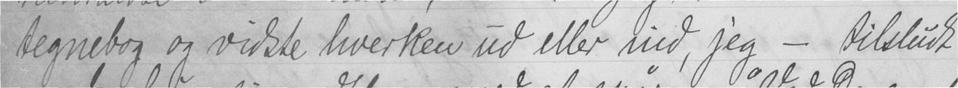tegnebog og vidste hverken ud eller ind, jeg - tilsludt
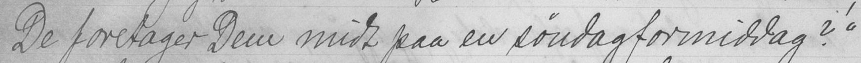De foretager Dem midt paa en søndagformiddag?"
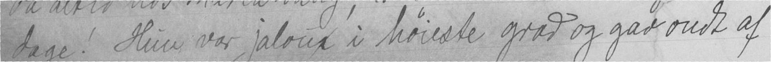dage! Hun var jalous i høieste grad og gav ondt af
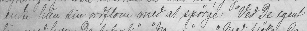endte hun sin ordflom med at spørge: "Ved De egent-
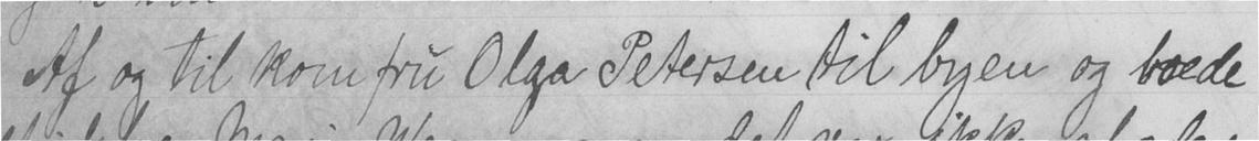Af og til kom fru Olga Petersen til byen og boede
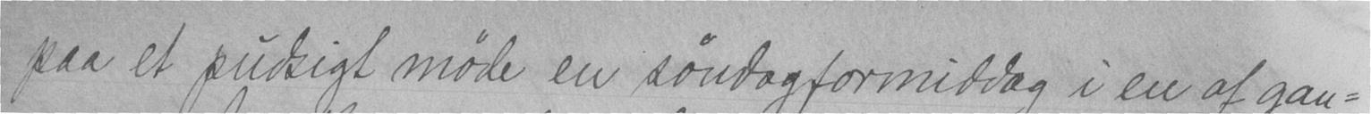paa et pudsigt møde en søndagformiddag i en af gan-
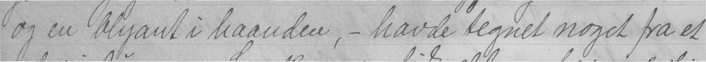og en blyant i haanden, - havde tegnet noget fra et
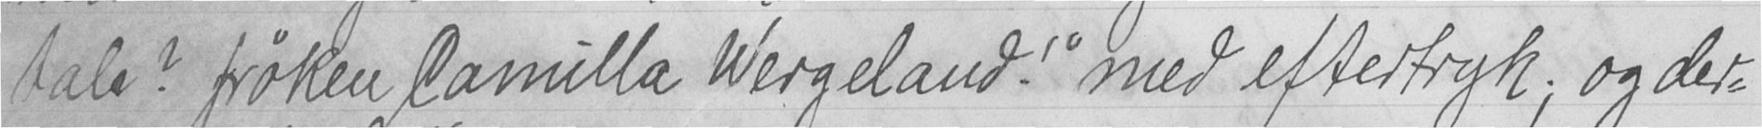tale? frøken Camilla Wergeland!" med eftertryk; og der-
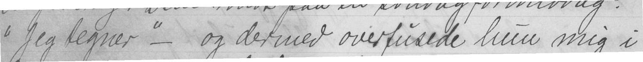"Jeg tegner" - og dermed overfusede hun mig i
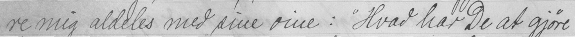re mig aldeles med sine øine: "Hvad har De at gjøre
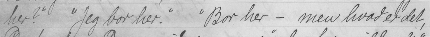her?" "Jeg bor her." "Bor her - men hvad er det,
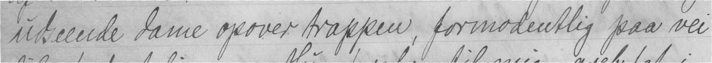udseende dame opover trappen, formodentlig paa vei
